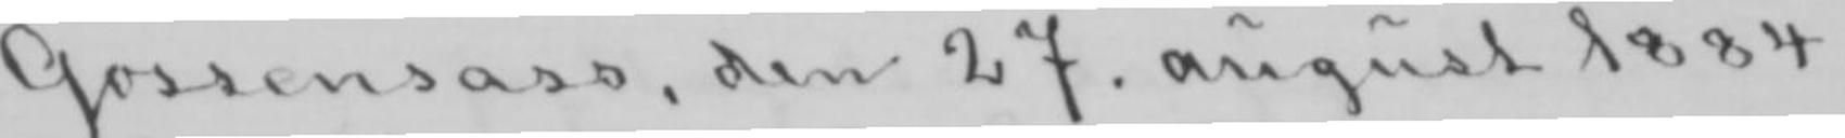Gossensass, den 27. august 1884.
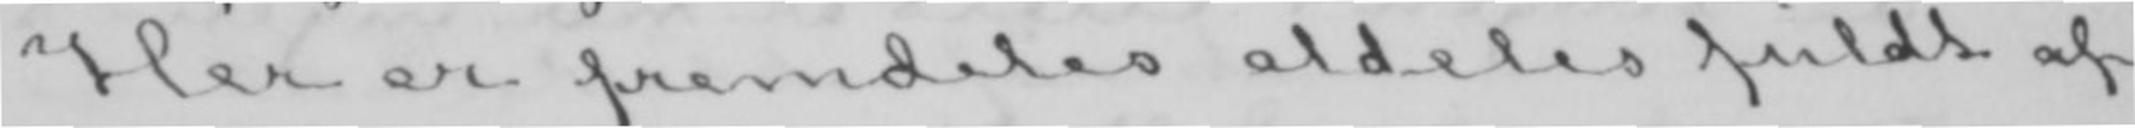Her er fremdeles aldeles fuldt af
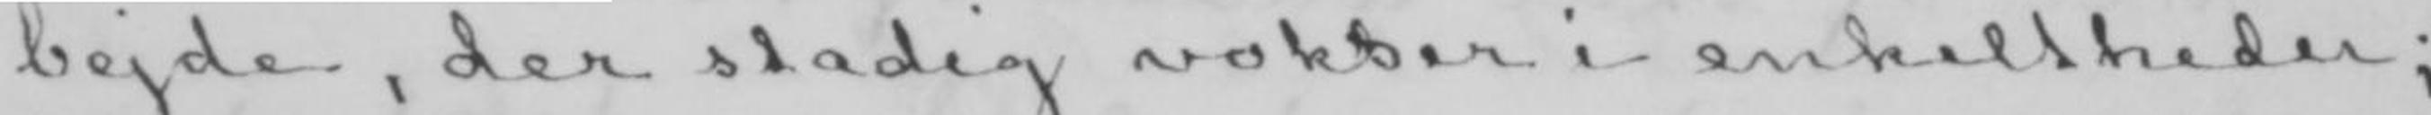bejde, der stadig vokser i enkeltheder;
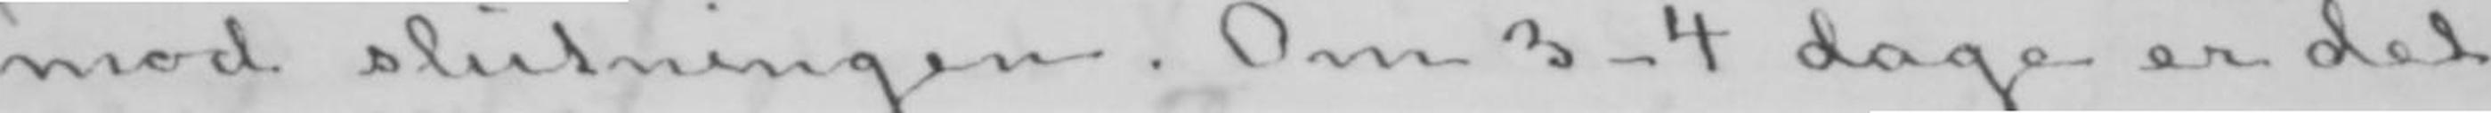mod slutningen. Om 3-4 dage er det
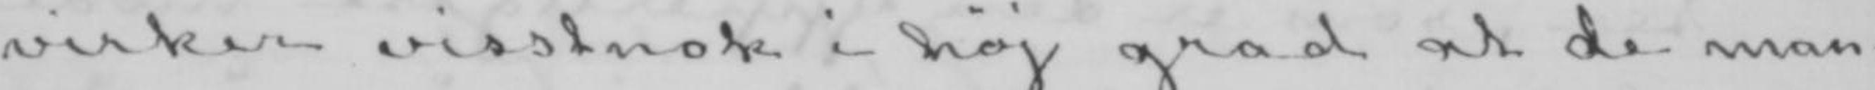virker visstnok i høj grad at de man-
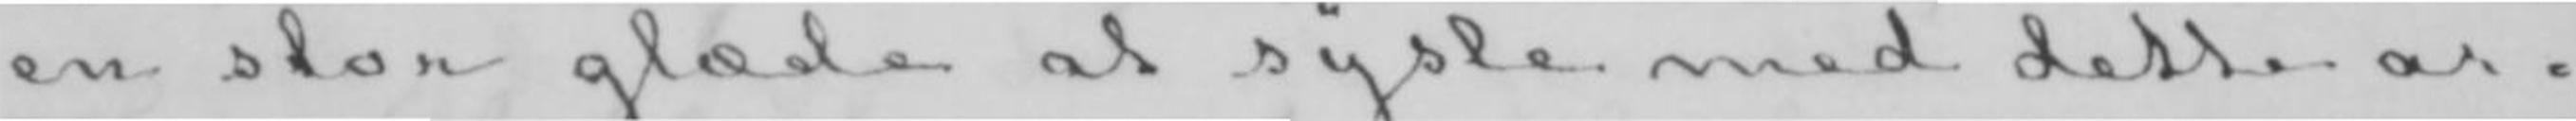en stor glæde at sysle med dette ar-
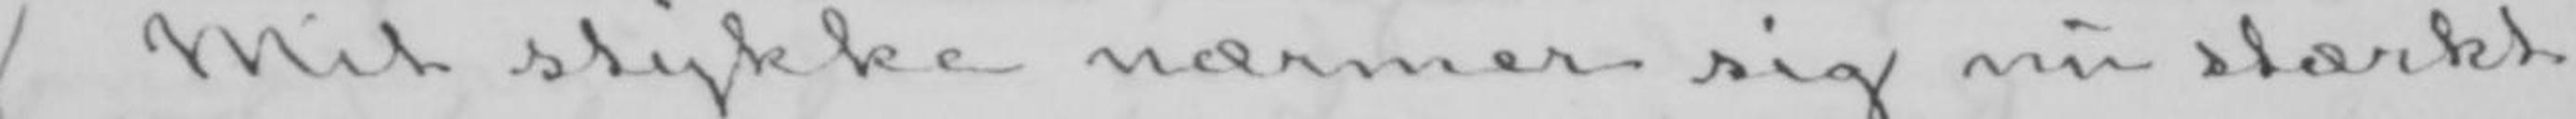Mit stykke nærmer sig nu stærkt
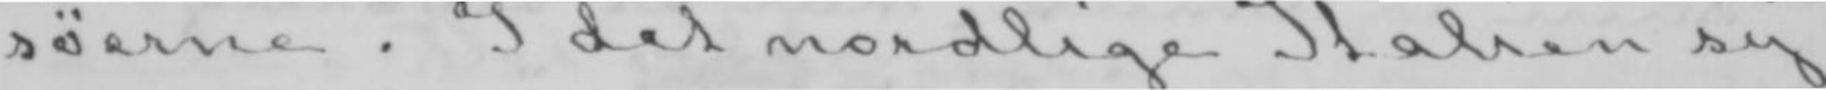søerne. I det nordlige Italien sy-
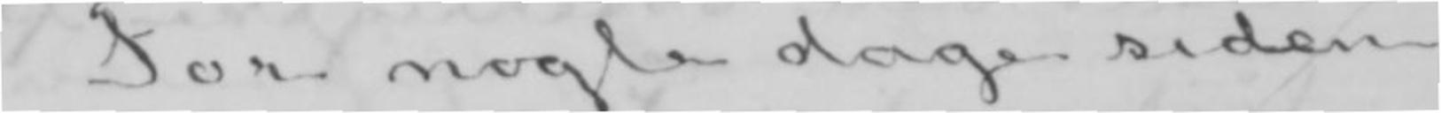For nogle dage siden
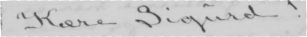Kære Sigurd!
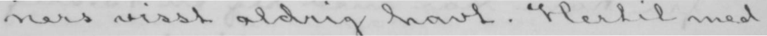ners visst aldrig havt. Hertil med-
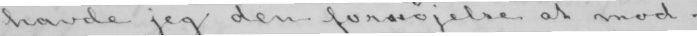havde jeg den fornøjelse at mod-
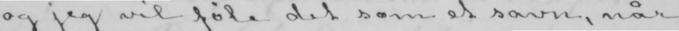og jeg vil føle det som et savn, når
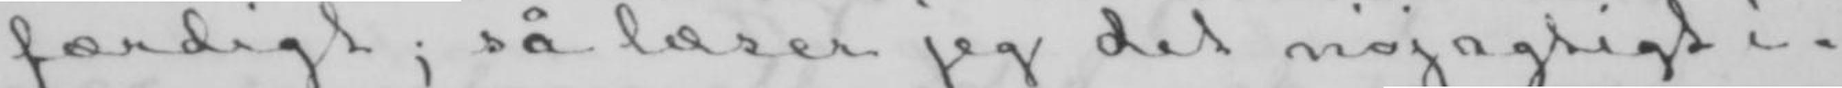færdigt; så læser jeg det nøjagtigt i-
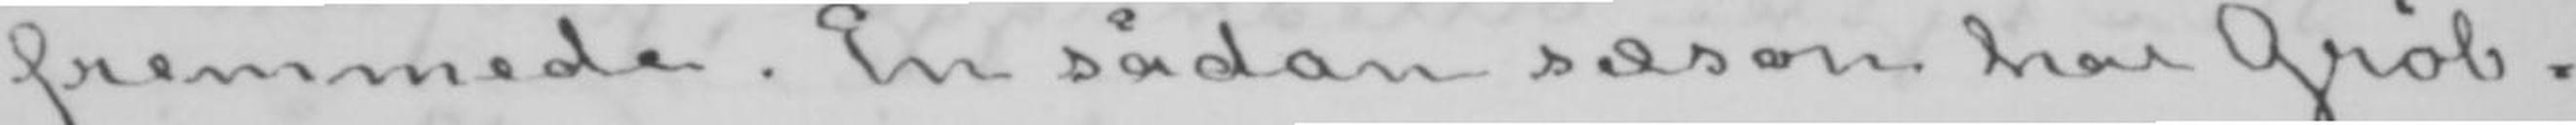fremmede. En sådan sæson har Gröb-
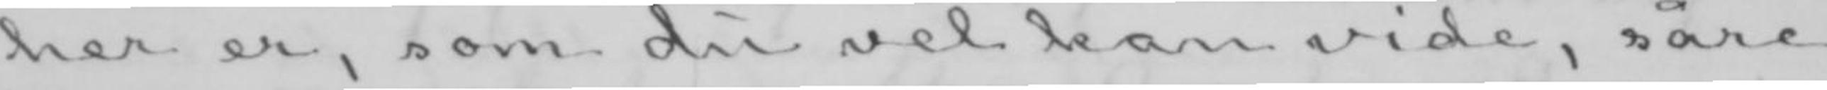her er, som du vel kan vide, såre
